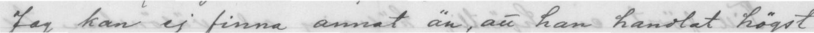Jag kan ej finna annat än, att han handlat högst
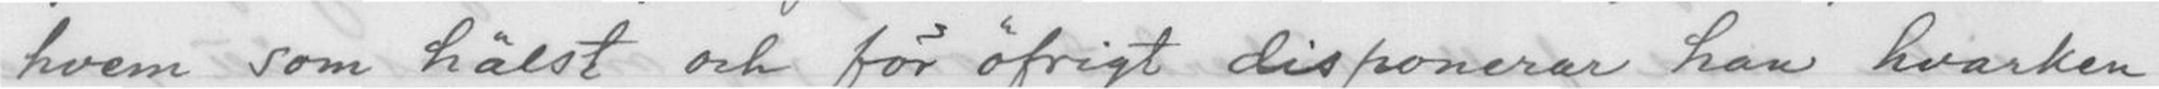hvem som hälst och för öfrigt disponerar han hvarken
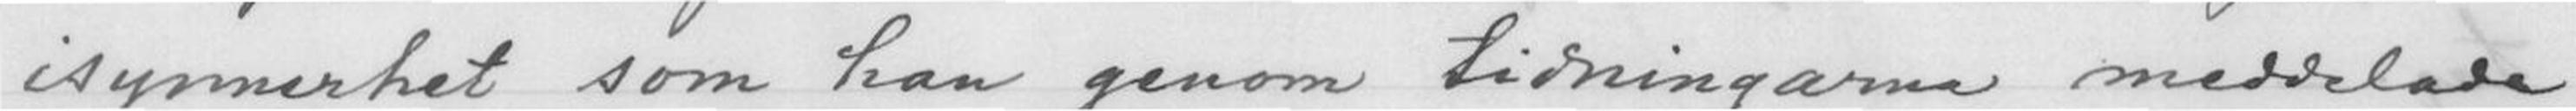isymmerhet som han genom tidningarna deddelade
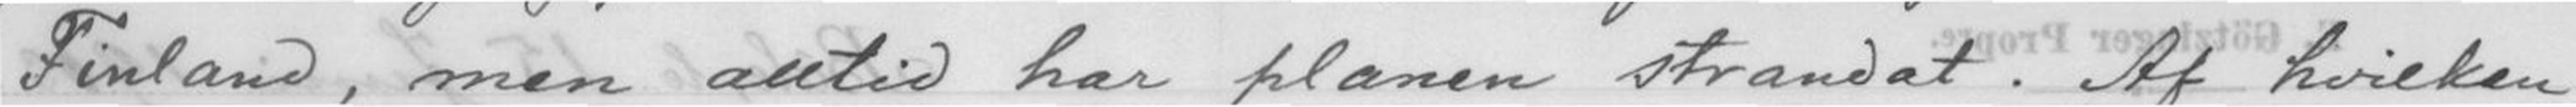Finland, men alltid har planen strandat. Af hvilken
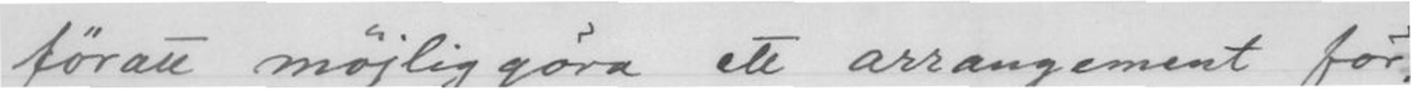föratt möjliggöra ett arrangement för
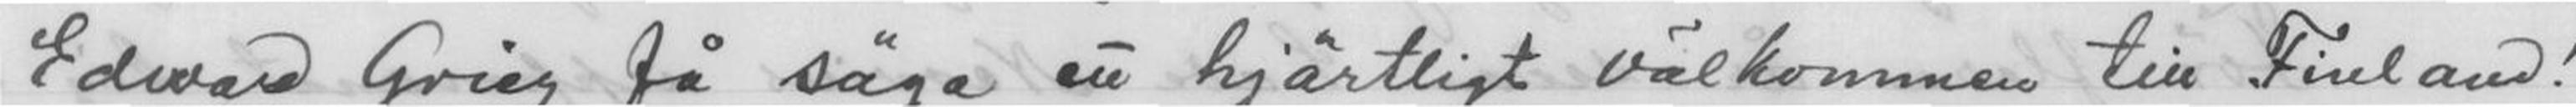Edward Grieg få säga ett hjärtligt välkommen till Finland!
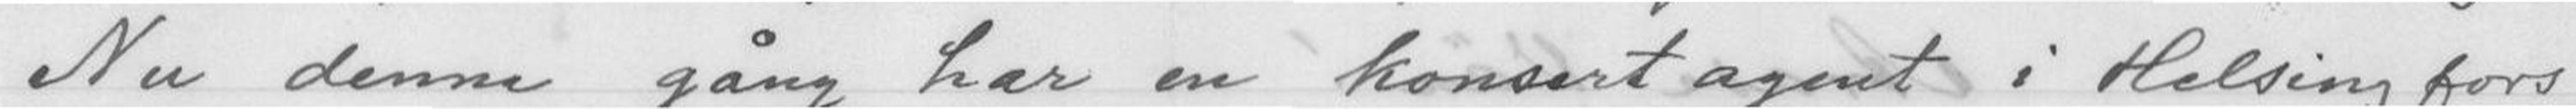Nu denne gång har en konsertagent i Helsingfors
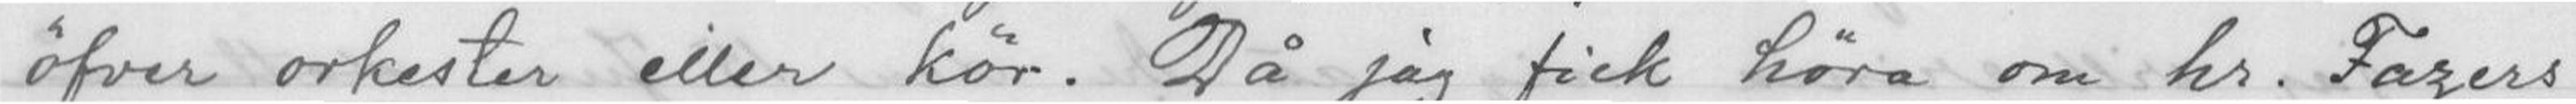öfver orkester eller kör. Då jag fick höra om hr. Fagers
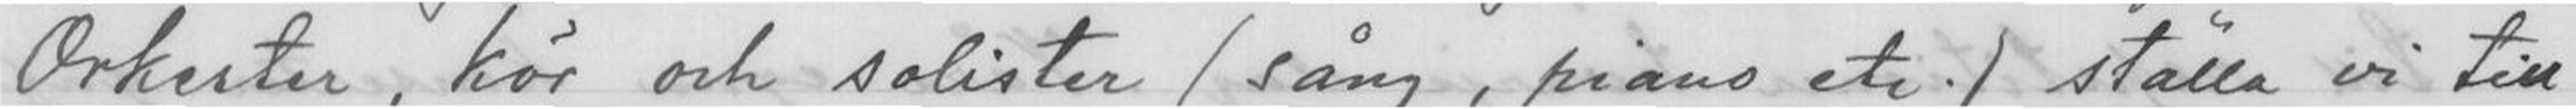Orkester, kör och solister (sång, piano etc.) ställa vi till
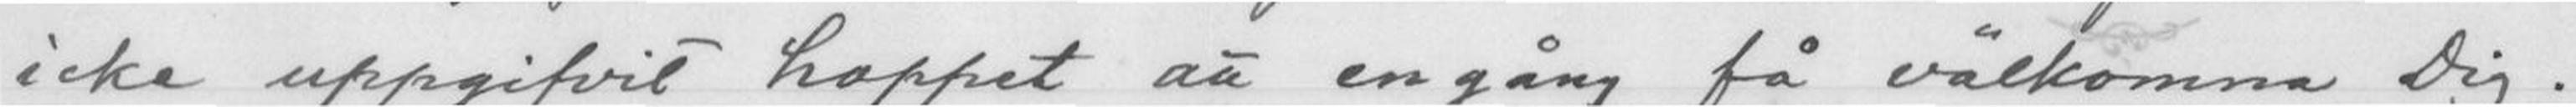icke uppgifvit hoppet att engång få välkomna Dig.
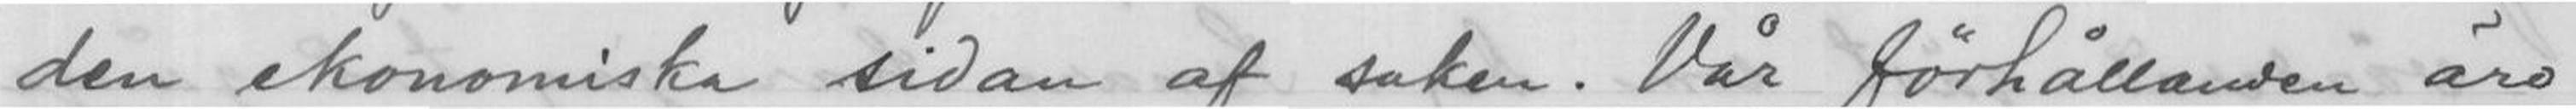den ekonomiska sidan af saken. Vår förhållanden ärs
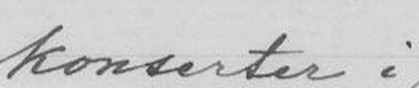konserter i
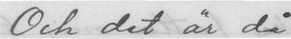Och det är då
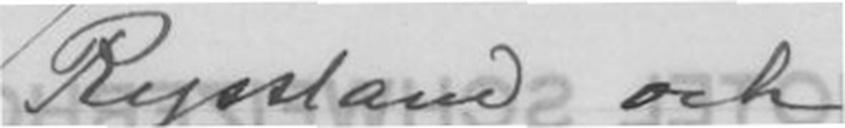Ryssland och
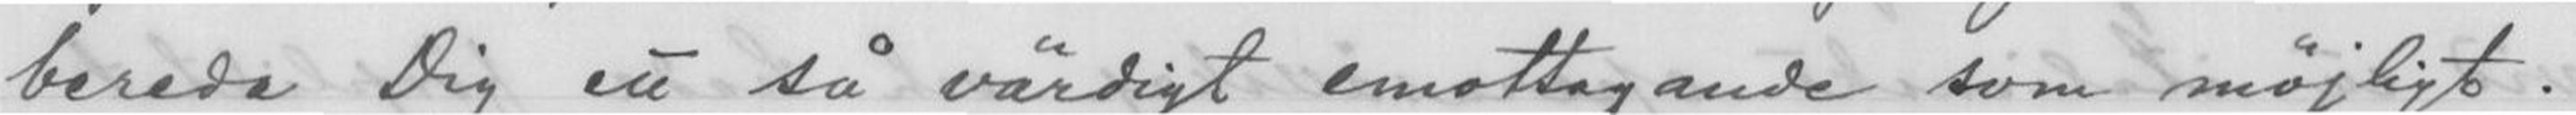bereda dig ett så värdigt emottagande som möjligt.
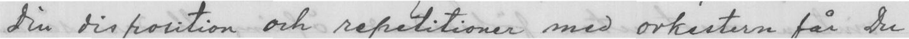din disposition och repetitioner med orkestern får Du
